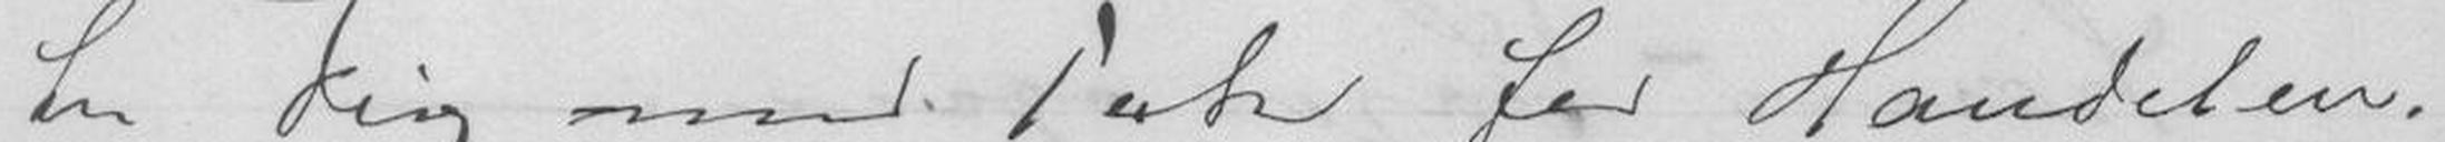til Dig med Tak for Handelen.
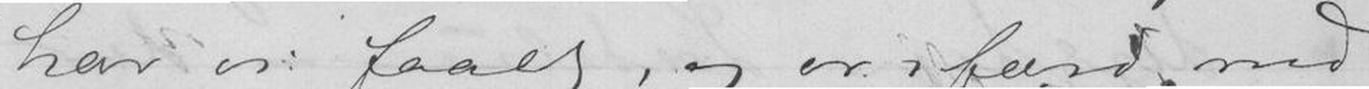har vi faaet, og er i færd med
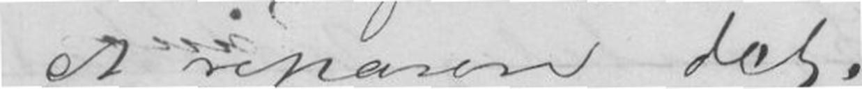at reparere det.
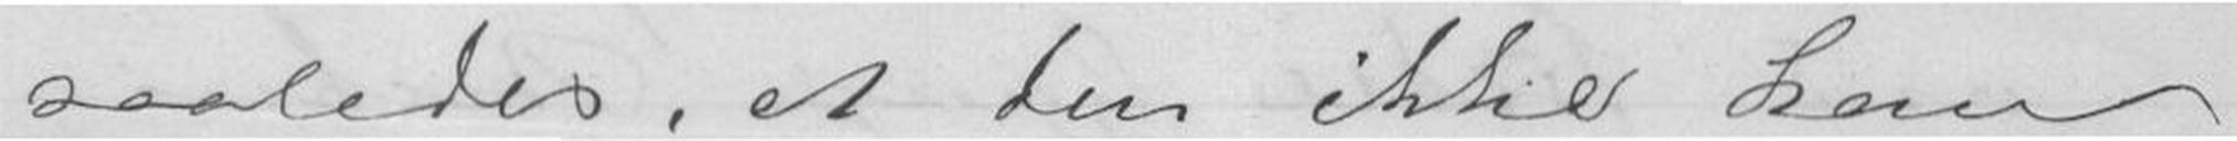saaledes, at den ikke kan
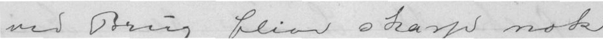ved Brug blive skarp nok
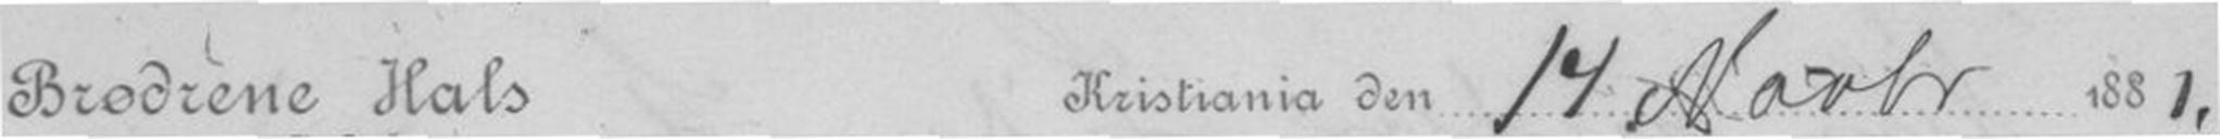Brødrene Hals Kristiania den 14 Novbr 1881.
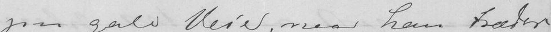paa gale Veier, naar han træder
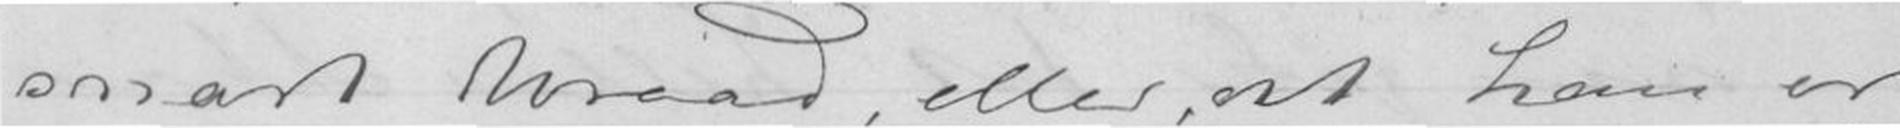snart Uraad, eller at han er
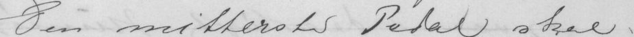Den mitterste Pedal skal
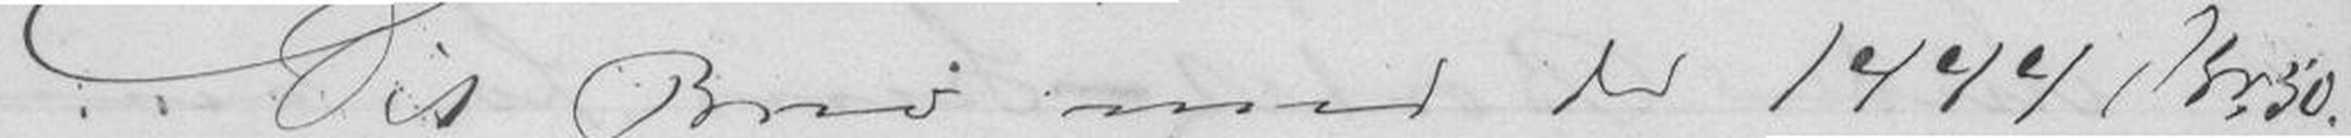Dit Brev med de 1444 Kr 50.
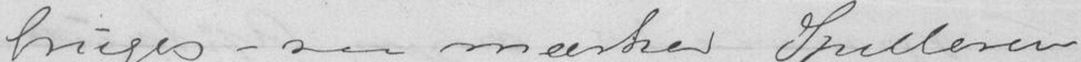bruges - saa mærker Spilleren
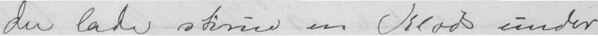Du lade skrue en Klods under
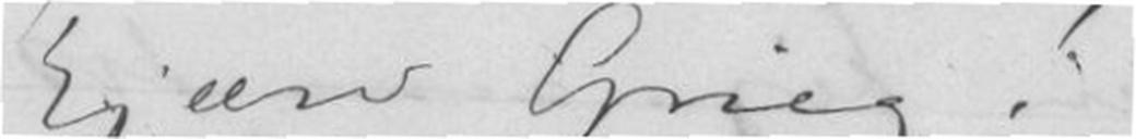Kjære Grieg!
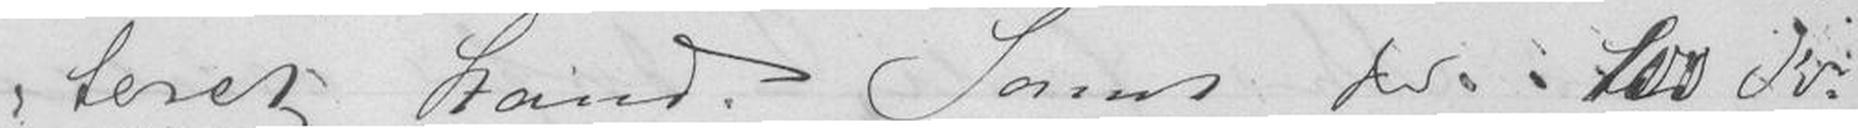teret Stand. Samt de 100 Kr.
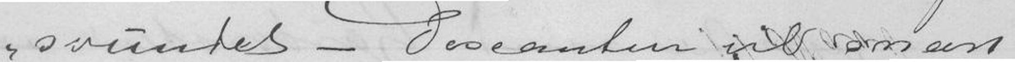svundet - Discanten vil snart
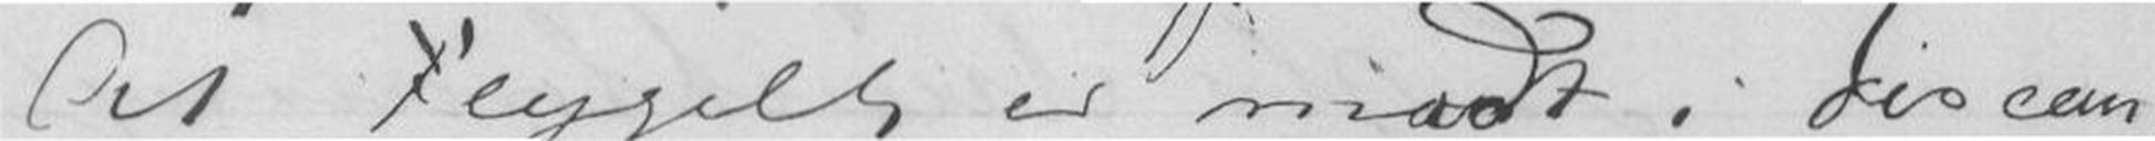At Flygelet er madt i discan
